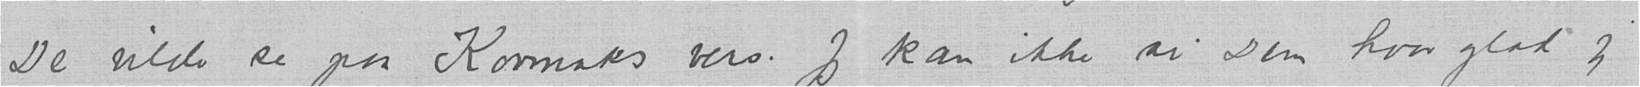De vilde se paa Kormaks vers. Jeg kan ikke si Dem hvor glad jeg
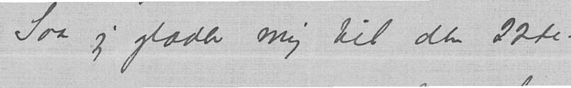Saa jeg glæder mig til den 22de.
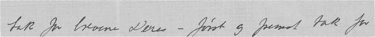tak for brevene Deres – først og fremst tak for
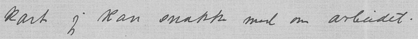kart jeg kan snakke med om arbeidet.
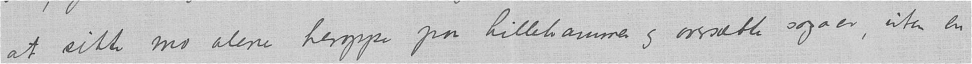at sitte mo alene heroppe paa Lillehammer og oversætte sagaer, uten en
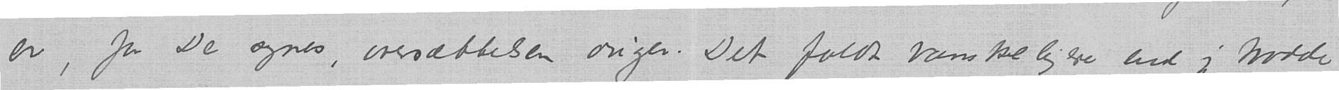er, for De synes oversættelsen duger. Det faldt vanskeligere end jeg trodde
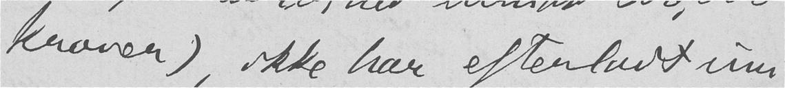kroner), ikke har efterladt uni-
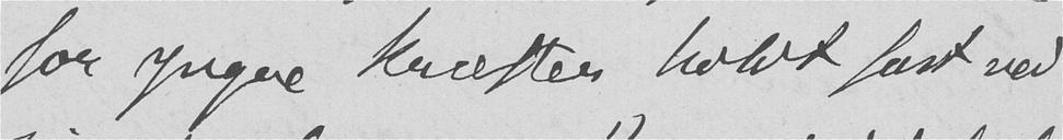for yngre kræfter holdt fast ved
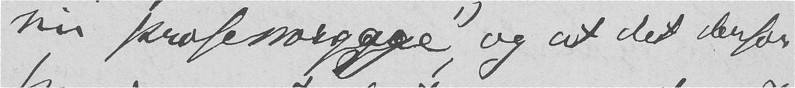sin professorgage 1), og at det derfor
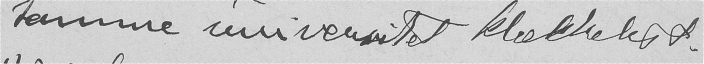samme universitet klækkeligt.
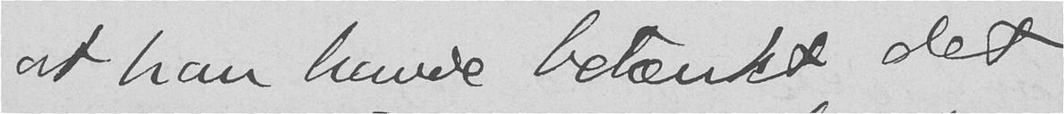at han havde betænkt det
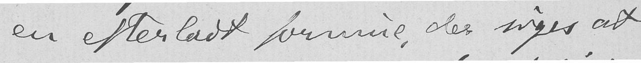en efterladt formue, der siges at
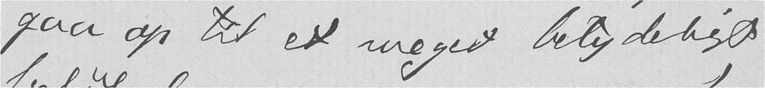gaa op til et meget betydeligt
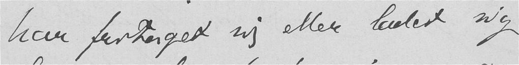har fritaget sig eller ladet sig
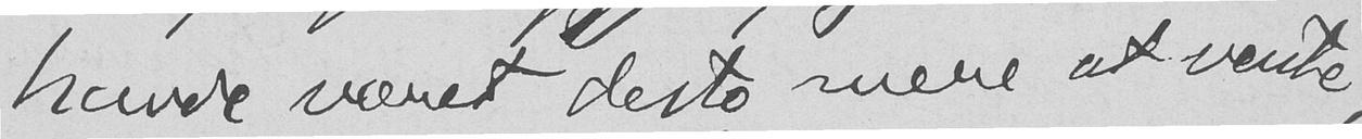havde været desto mere at vente,
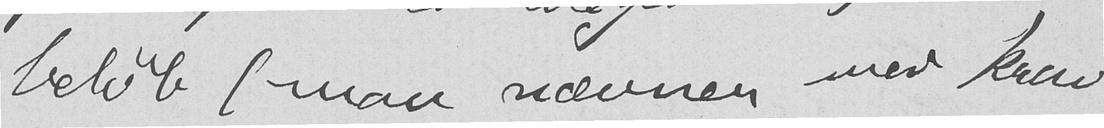beløb (man nævner med krav
